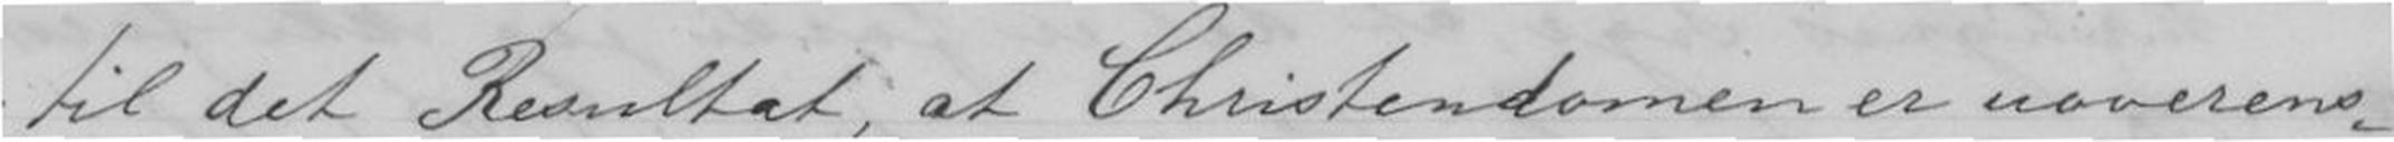til det Resultat, at Christendomen er uoverens-
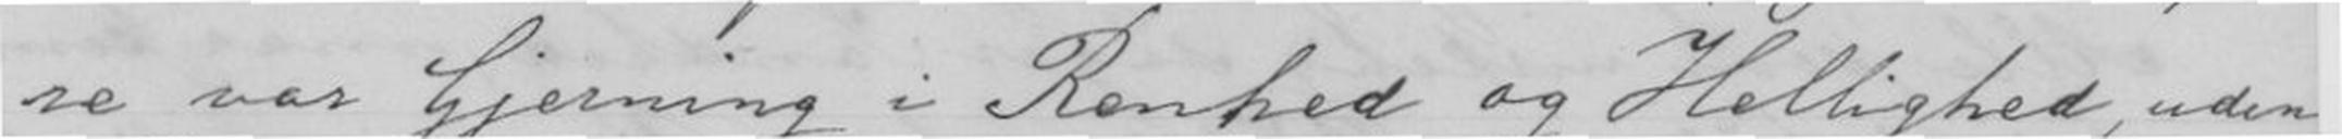re vor Gjerning i Renhed og Hellighed, uden
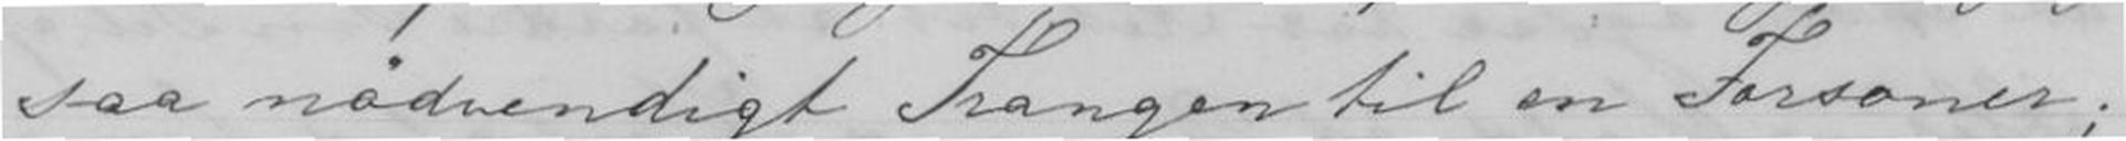saa nødvendigt Trangen til en Forsoner;
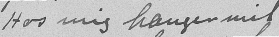Hos mig hænger mit
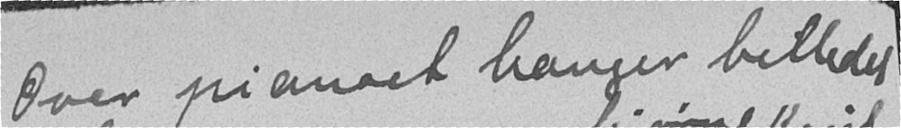Over pianoet hænger billedet
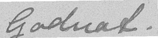Godnat!
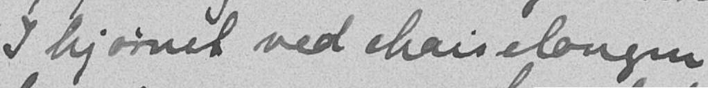I hjørnet ved chaiselongen
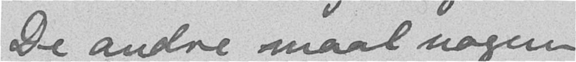De andre maal nogen-
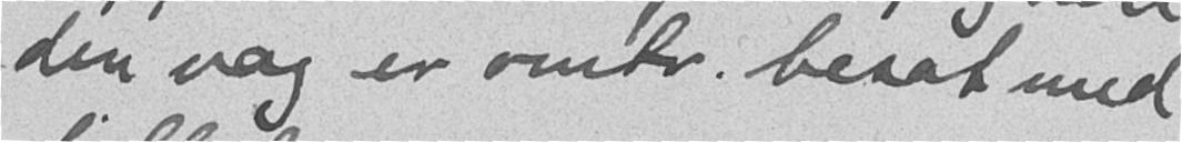den væg er omtr. besat med
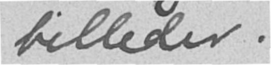billeder.
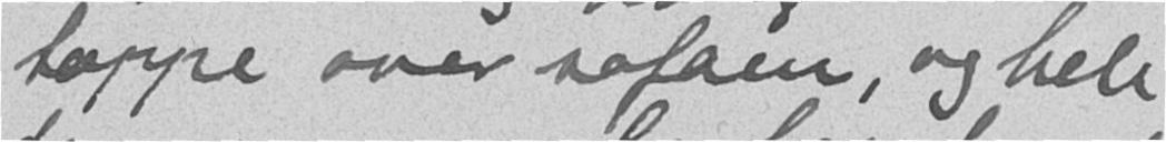tæppe over sofaen, og hele
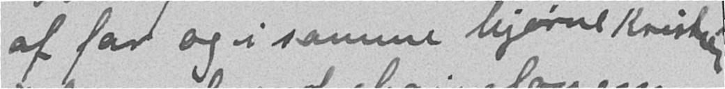af far og i samme hjørne Kristine
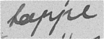tæppe.
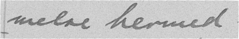melse hermed.
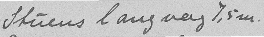Stuens langvæg 7,5 m.
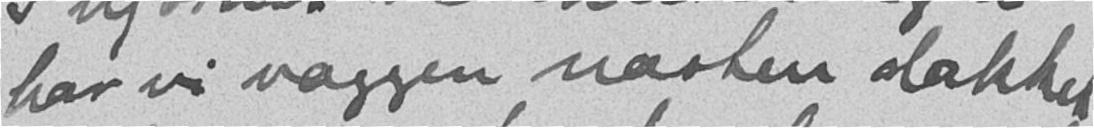har vi væggen næsten dækket
